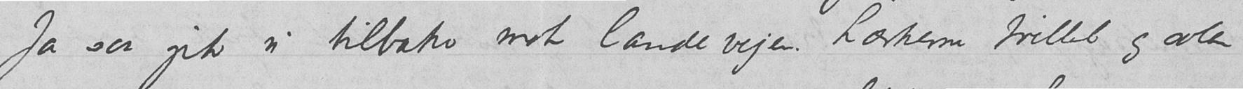Ja saa gik vi tilbake mot landevejen. Lærkerne trillet og solen
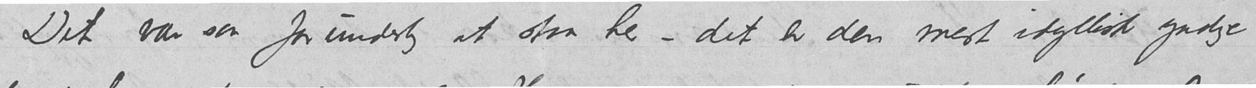Det var saa forunderlig at staa her – det er den mest idyllisk yndige
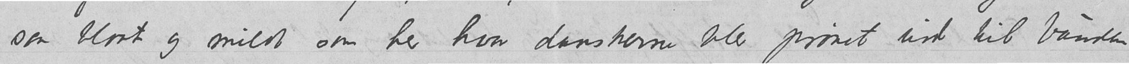saa blaat og mildt som her hvor danskerne blev prøvet ind til bunden
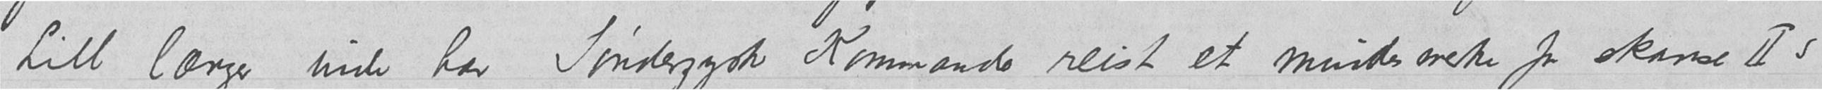Litt længer inde har Sønderjysk Kommando reist et mindesmerke for skanse lls
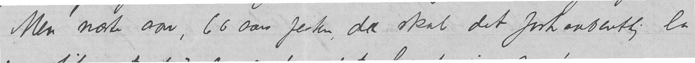Men næste aar, 60 aars festen, da skal det forhaabentlig la
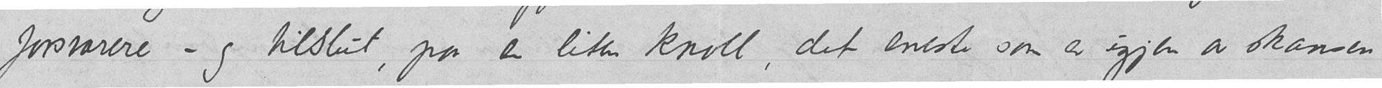forsvarere – og tilslut, paa en liten knoll, det eneste som er igjen av skansen
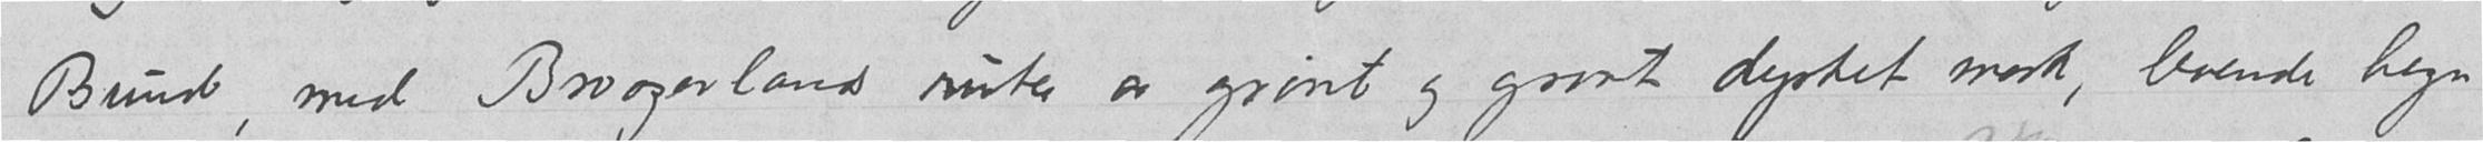Bund, med Broagerlands ruter av grønt og graat dyrket mark, levende hegn
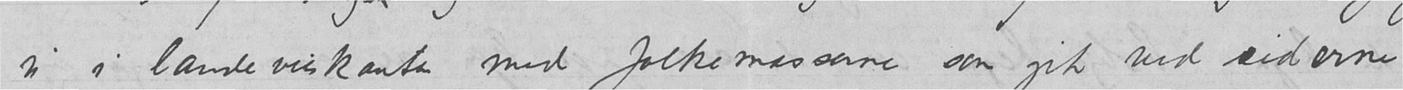vi i landeveiskanten med folkemasserne som gik ved siderne
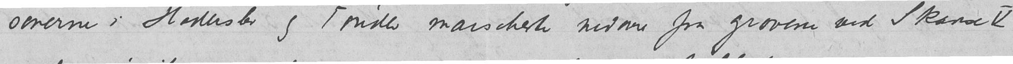sonerne i Haderslev og Tønder marscherte nedover fra gravene ved Skanse V
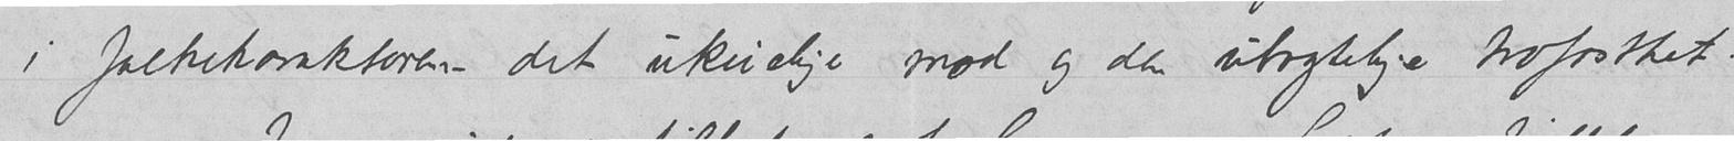i folkekarakteren – det ukuelige mod og den ubrytelige trofasthet.
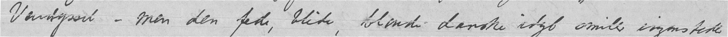Vendsyssel – men den fede, blide, blonde danske idyl smiler ingensteder
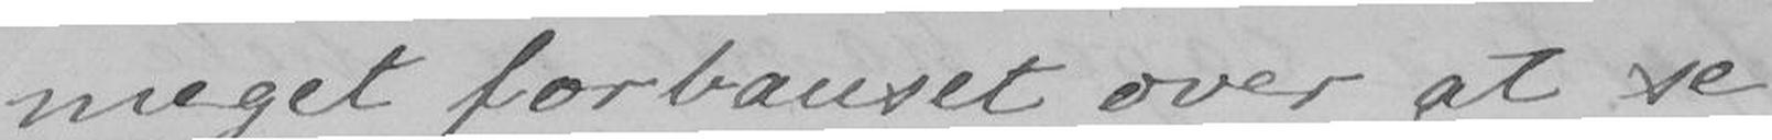meget forbauset over at se
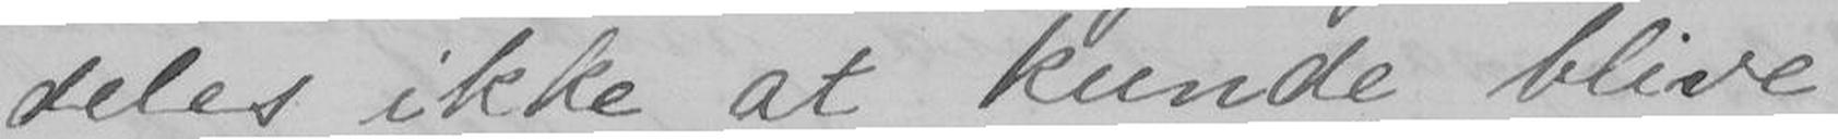deles ikke at kunde blive
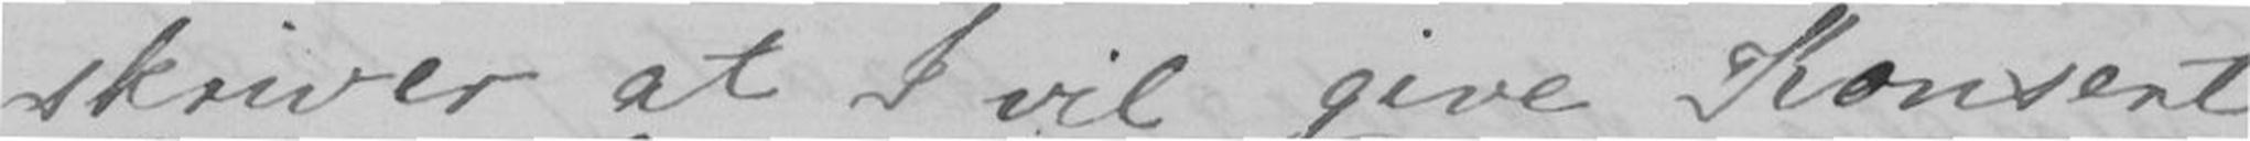skriver at I vil give Konsert
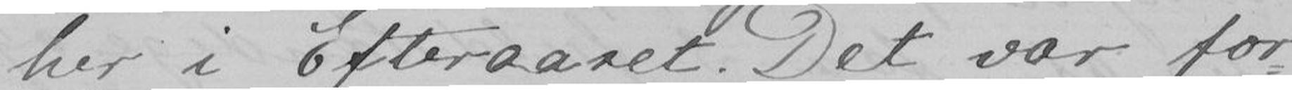her i Efteraaret. Det var for-
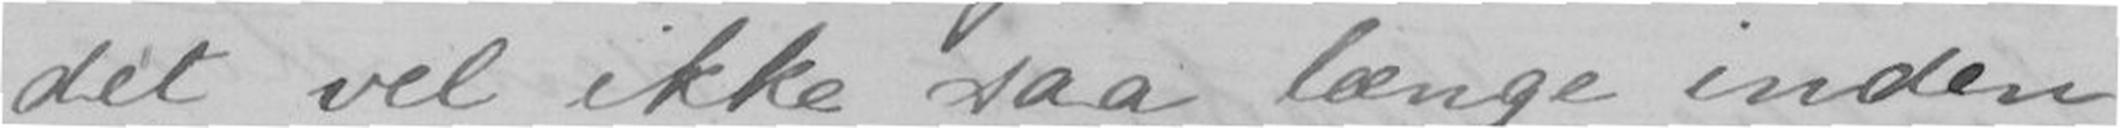det vel ikke saa længe inden
